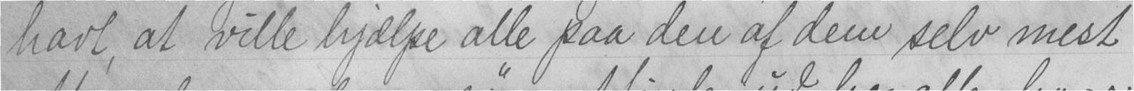havt, at ville hjælpe alle paa den af dem selv mest
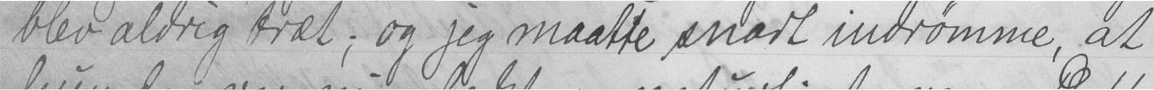blev aldrig træt; og jeg maatte snart indrømme, at
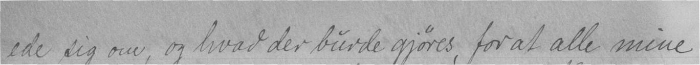ede sig om, og hvad der burde gjøres, for at alle mine
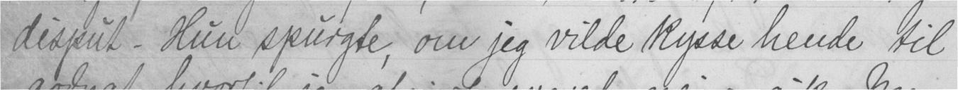disput. Hun spurgte, om jeg vilde kysse hende til
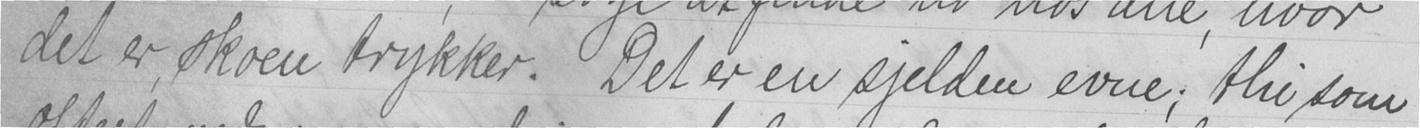det er skoen trykker. Det er en sjelden evne; thi som
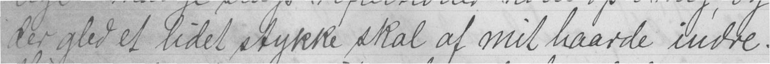der gled et lidet stykke skal af mit haarde indre.
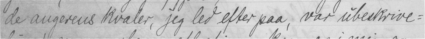de angerens kvaler, jeg led efter paa, var ubeskrive-
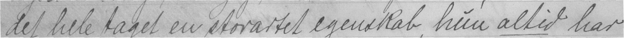det hele taget en storartet egenskab, hun altid har
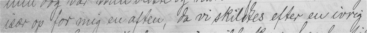især op for mig en aften, da vi skildtes efter en ivrig
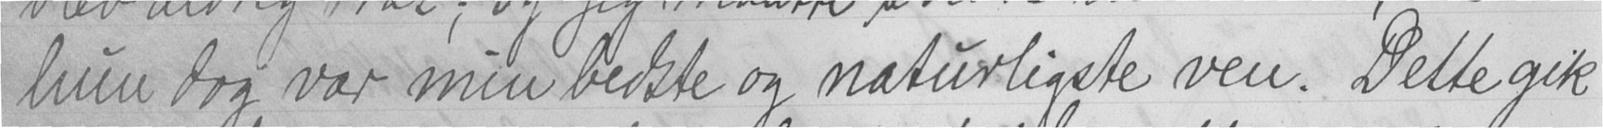hun dog var min bedste og naturligste ven. Dette gik
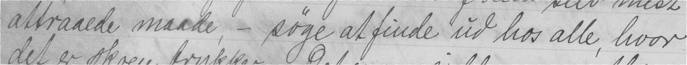attraaede maade, - søge at finde ud hos alle, hvor
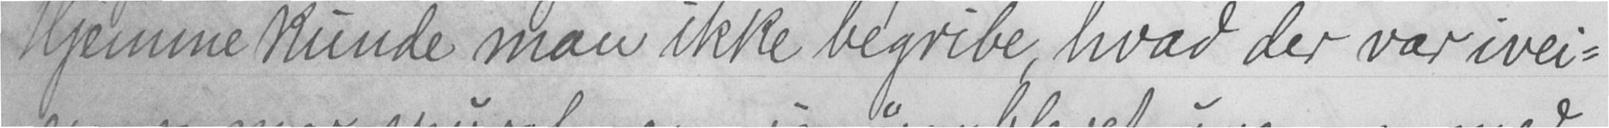Hjemme kunde man ikke begribe hvad der var ivei-
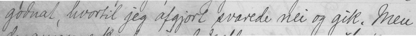godnat, hvortil jeg afgjørt svarede nei og gik. Men
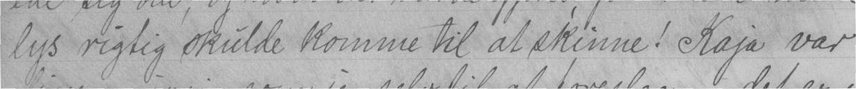lys rigtig skulde komme til at skinne! Kaja var
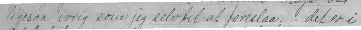ligesaa ivrig som jeg selv til at foreslaa; - det er i
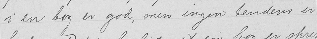i en bog er god, men ingen tendens er
